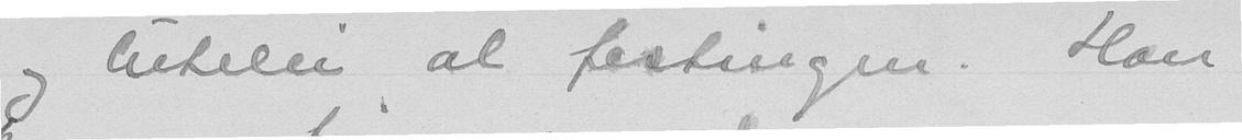og lutelei al festingen. Han
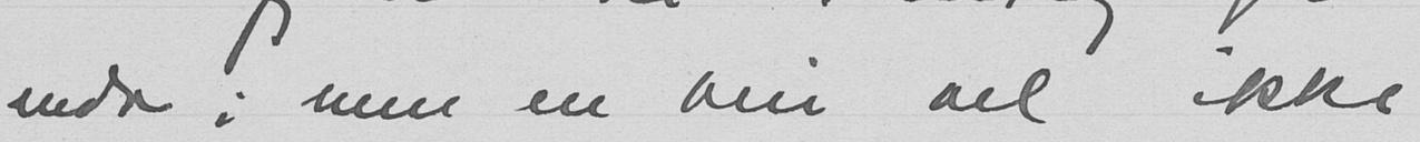enda; men en blir vel ikke
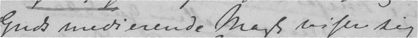Guds medierende Magt viser sig i
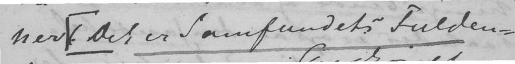ner. Det er Samfundets Fulden-
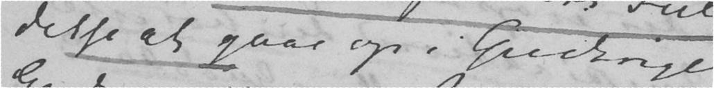delse at gaae op i Gudsriget.
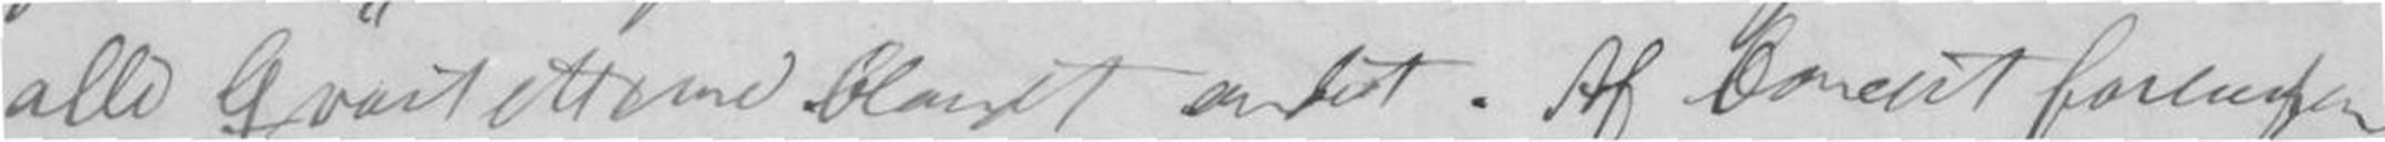alle qvartettene blandt andet. Af Concert foreningen
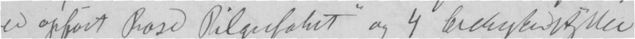er opført Rose Pilgerfahrt og 4 Orchesterstykke
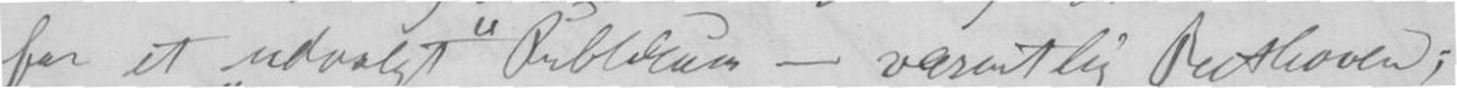for et udvalgt Publikum - væsentlig Beethoven;
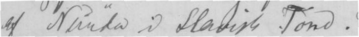af Neruda i Slavisk Tone.
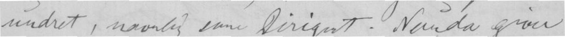undret, navnlig som Dirigent. Neruda giver
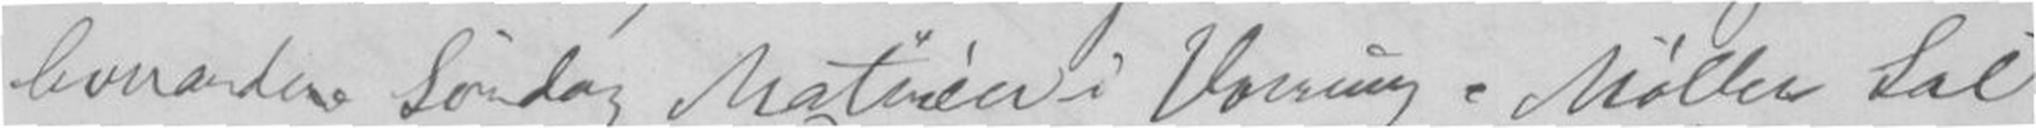Concert Søndag Matinéer i Hornung=Møllers Sal
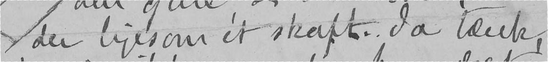der ligesom et skaft. Ja tænk,
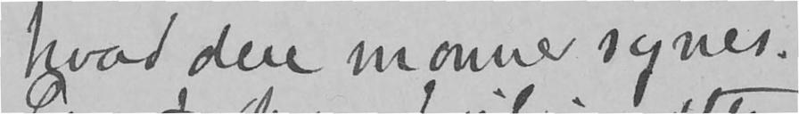hvad dere monne synes.
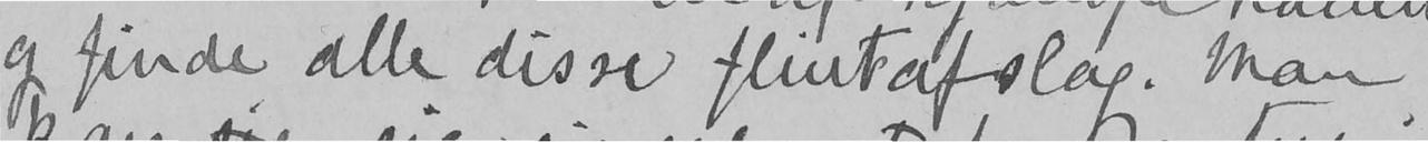og finde alle disse flintafslag. Man
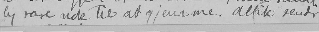lig rare nok til at gjemme. Allik sender
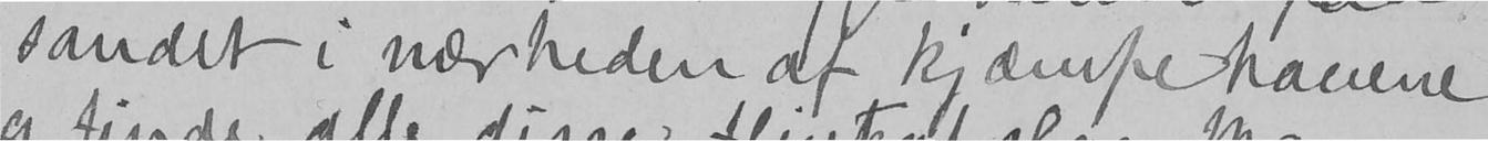sandet i nærheden af kjæmpehavene
